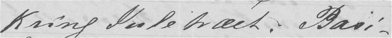kring Juletræet. Basi-
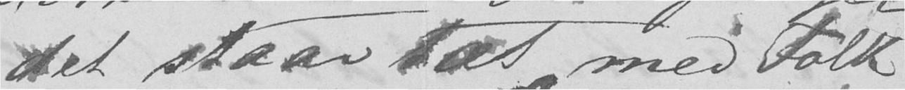det staar tæt med Folk
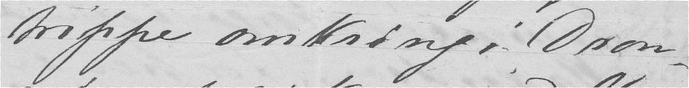trippe omkring i Dron-
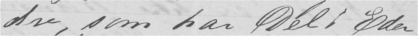dre, som har Del i Eder.
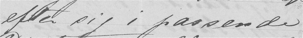efter sig i passende
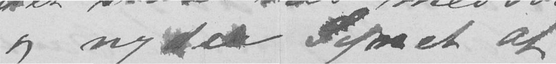og nyder Synet af
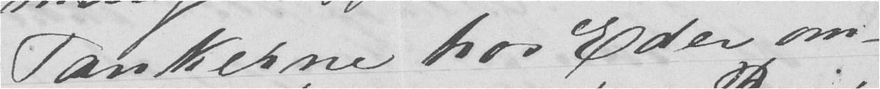Tankerne hos Eder om-
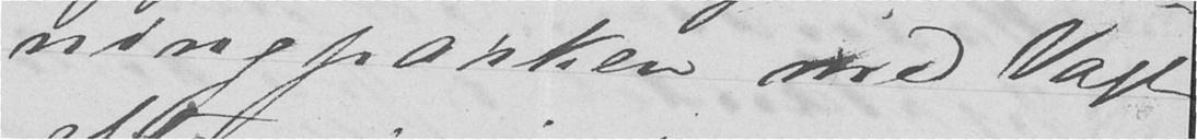ningparken med Vagt
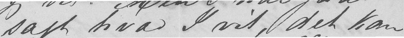sagt hvad I vil, det kan
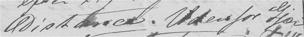Distance. Udenfor Gjær-
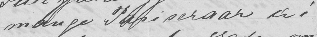mange Pariseraar er i
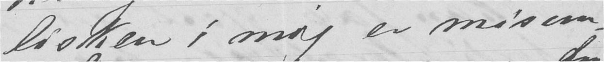lisken i mig er misun-
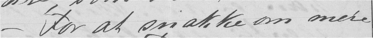- For at snakke om mere
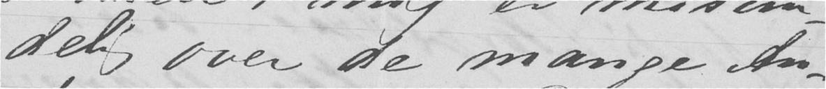delig over de mange An-
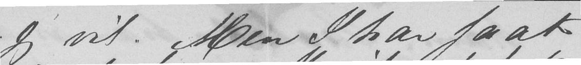jeg vil. Men I har faat
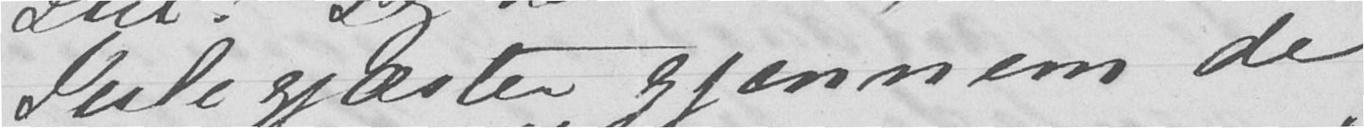Julegjæster gjennem de
